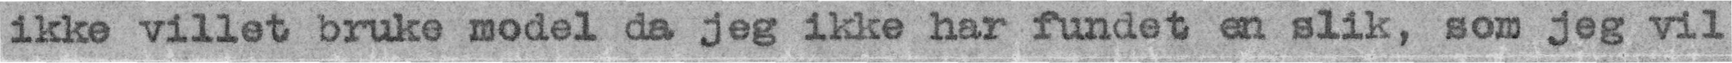ikke villet bruke model da jeg ikke har fundet en slik, som jeg vil
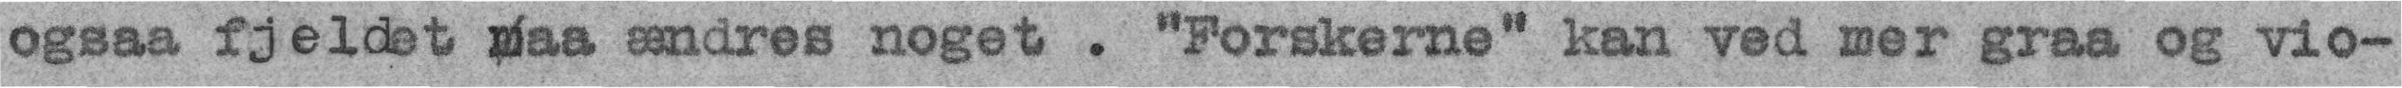ogsaa fjeldet maa ændres noget. «Forskerne» kan ved mer graa og vio-
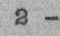– 2 –
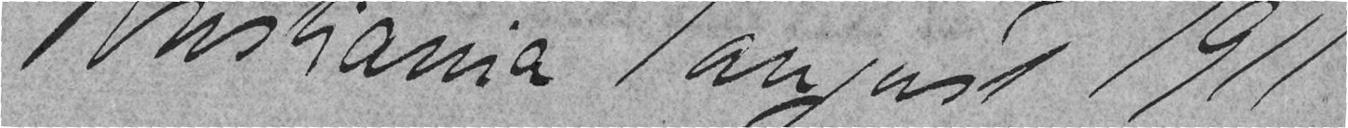Kristiania 1 august 1911
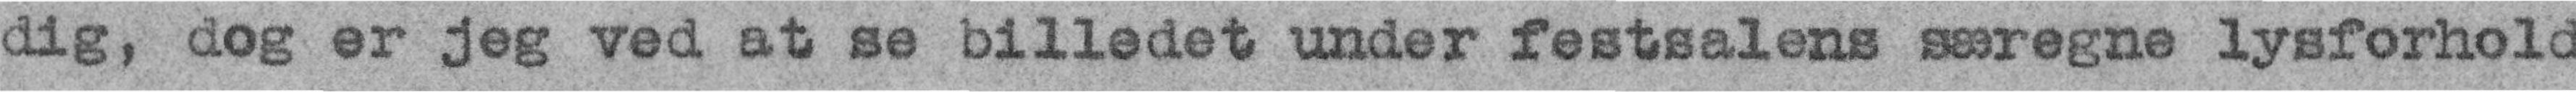dig, dog er jeg ved at se billedet under festsalens særegne lysforhold
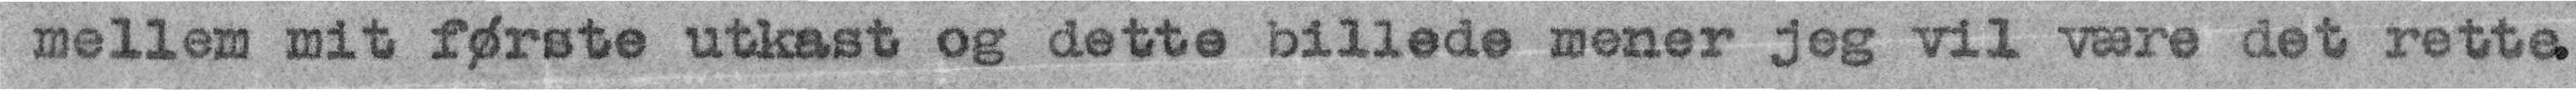mellem mit første utkast of dette billede mener jeg vil vil være det rette
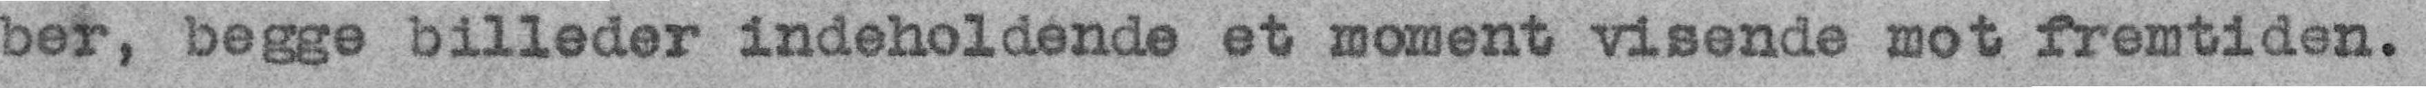ber, begge billeder indeholdende et moment visende mot fremtiden.
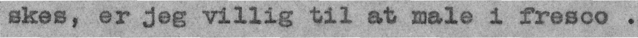skes, er jeg villig til at male i fresco.
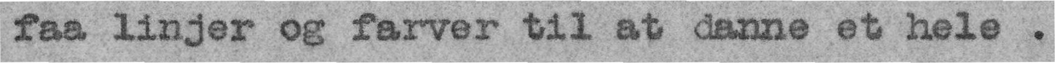faa linjer og farver til at danne et hele.
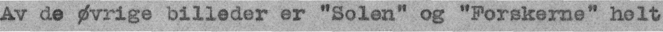Av de øvrige billeder er «Solen» og «Forskerne» helt
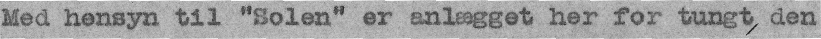Med hensyn til «Solen» er anlægget her for tungt, den
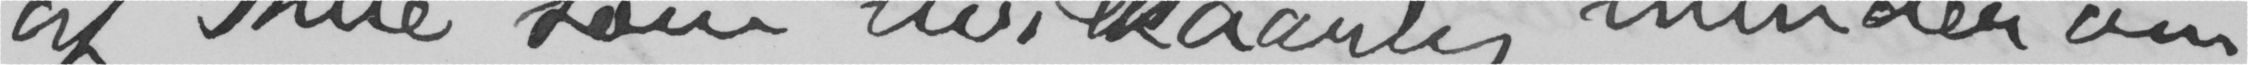af Thue som uvilkaarlig minder om
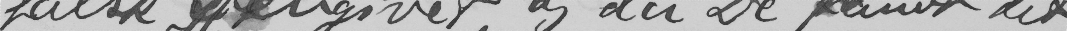falsk gjengivet, og da De fandt det
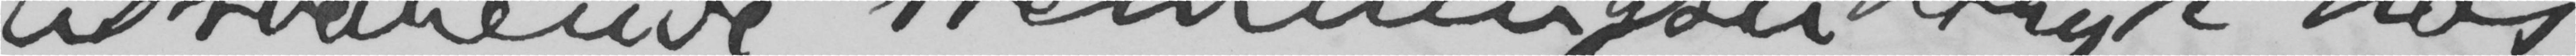tilsvarende stemningsudtryk hos
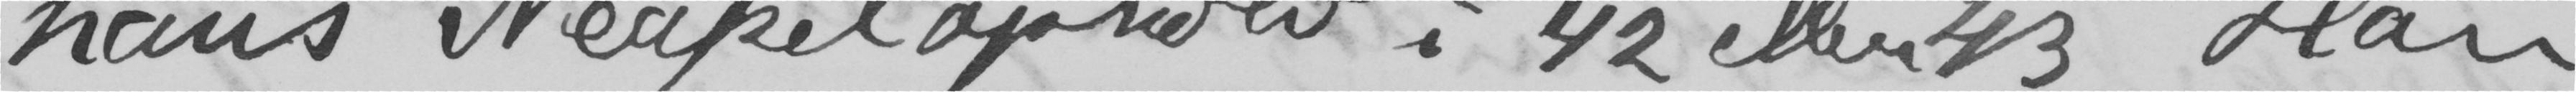hans Neapelophold i 42 eller 43. Han
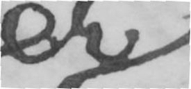er
